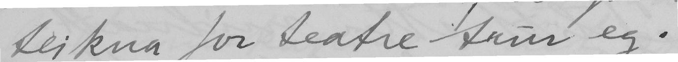teikna for teatre trur eg.
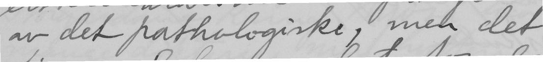av det pathologiske, men det
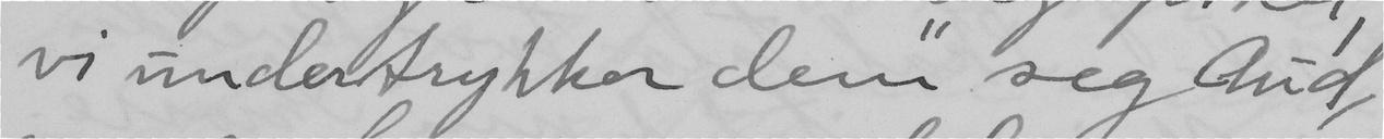vi undertrykker dem" seg Aud,
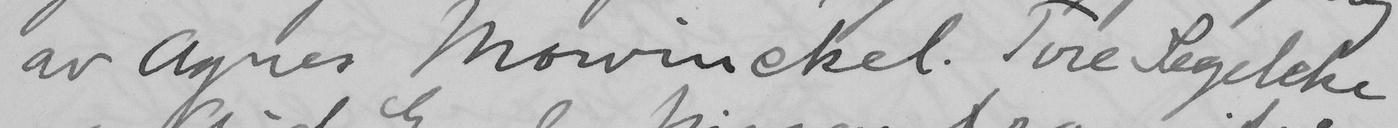av Agnes Mowinckel. Tore Segelcke
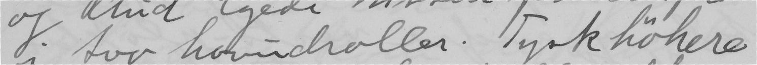i tvo hovudroller. Tysk höhere
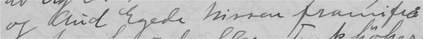og Knud Egede Nissen framifrå
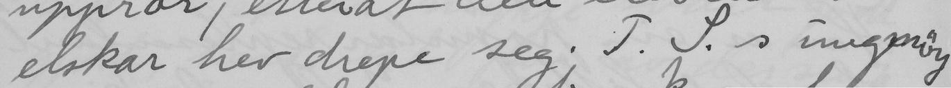elskar hev drepe seg. T. S.s ungmöy
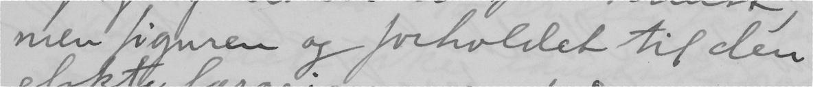men figuren og forholdet til den
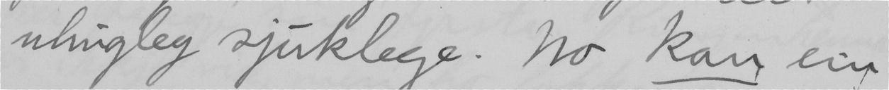utrugleg sjuklege. No kan ein
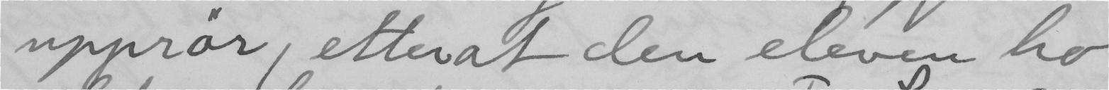upprör, etterat den eleven ho
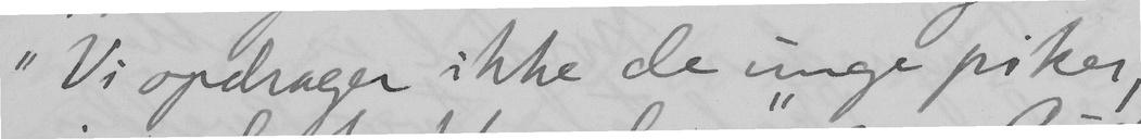"Vi opdrager ikke de unge piker,
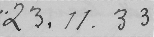23.11.33
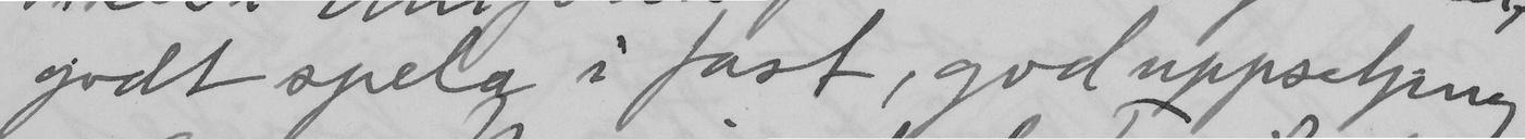godt spela i fast, god uppsetjing
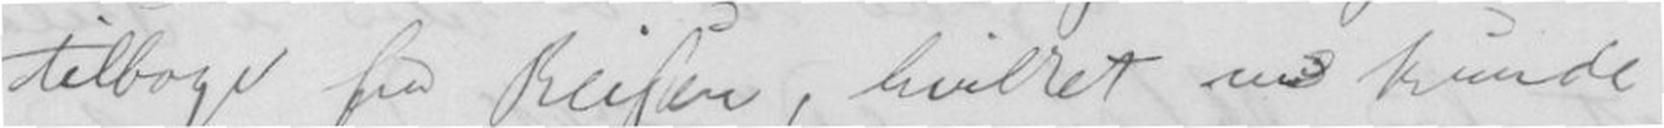tilbage fra Reisen, hvilket nu kunde
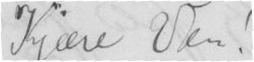Kjære Ven!
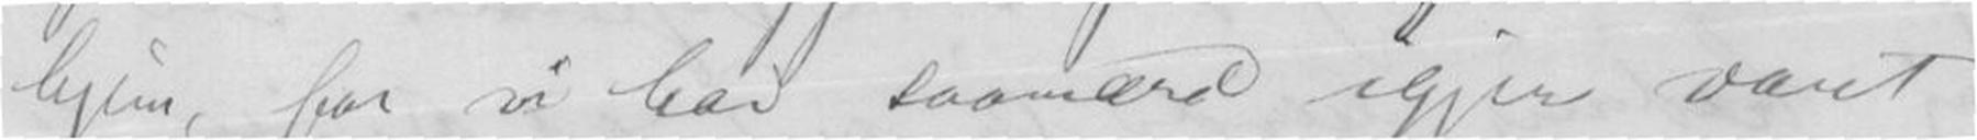hjem, for vi har saamænd. igjen vært
